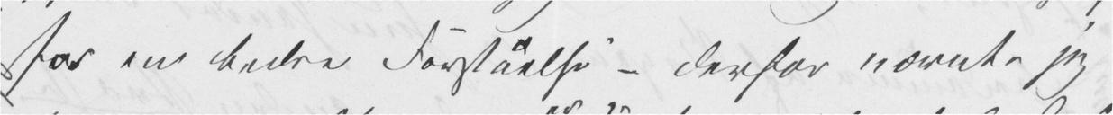for en bedre Forståelse – derfor nævnte jeg
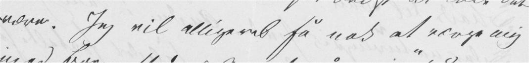være. Jeg vil alligevel få nok at værge mig
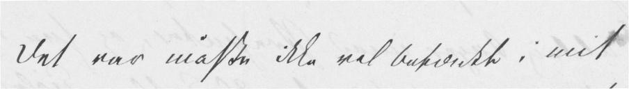Det var måske ikke vel betænkt i mit
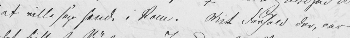at ville søge hende i Rom. Mit Forhold der, var
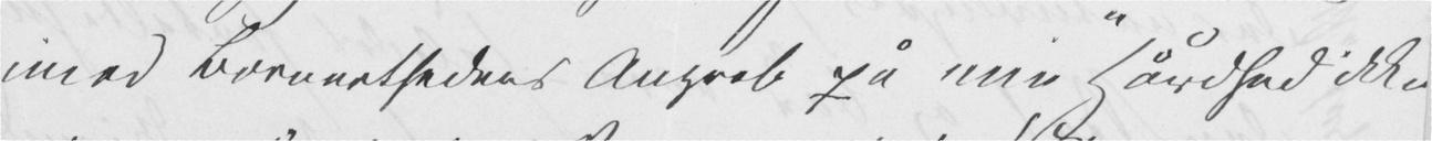imod Bornerthedens Angreb på min "Hårdhed ikke
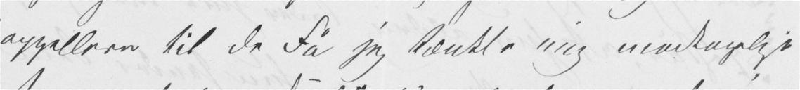appellere til de Få jeg tænkte mig modtagelige
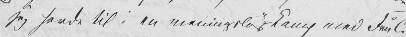jeg havde til i en meningsløs Kamp med Fru C.
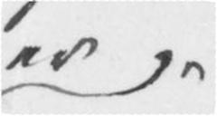er
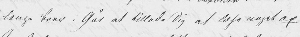lange Brev i Går at tillade Dig at læse noget op
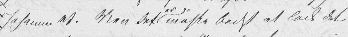Johanne V. Men det måske bedst at lade det
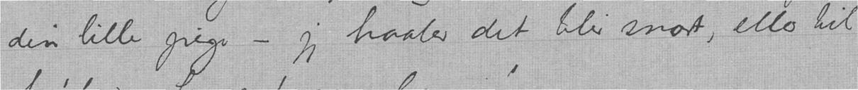din lille pige — jeg haaber det blir snart, eller til
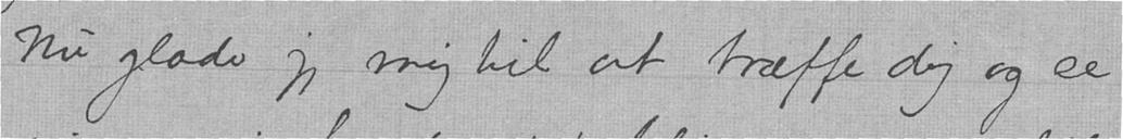Nu glæder jeg mig til at treffe dig og se
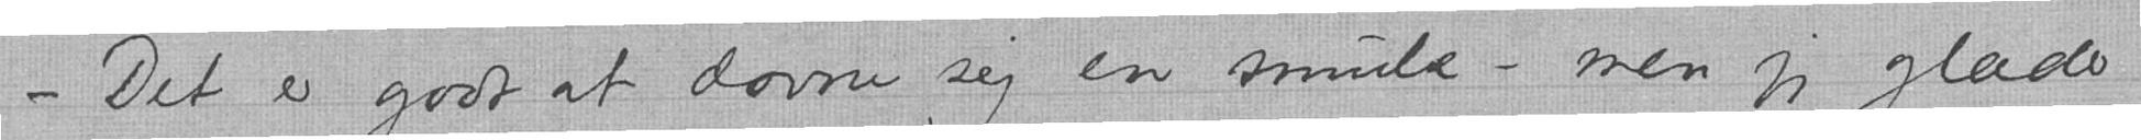— Det er godt at dovne sig en smule — men jeg glæder
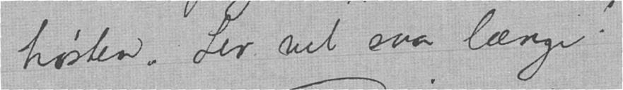høsten. Lev vel saa længe!
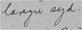længre syd.
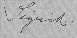Sigrid.
